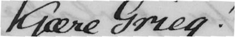Kjære Grieg!
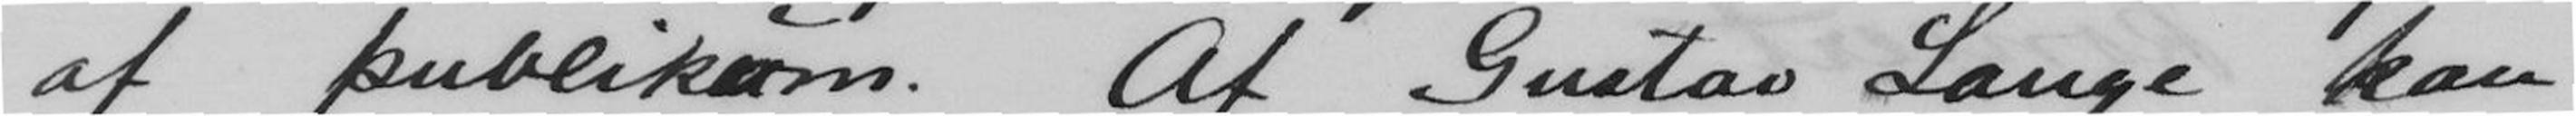af publikum. Af Gustav Lange kan
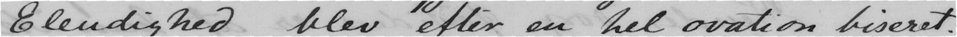Elendighed blev efter en hel ovation biseret.
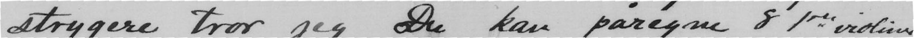strygere tror jeg Du kan påregne 8 1ste violine,
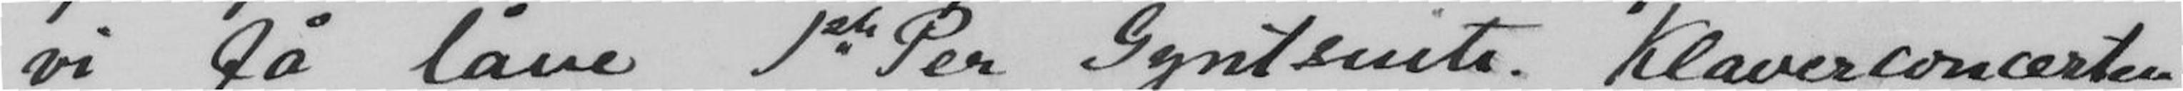vi få låne 1ste Per Gyntsuite. Klaverconcerten
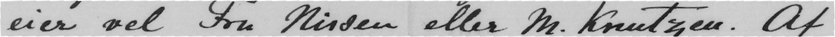eier vel Fru Nissen eller M. Knutzen. Af
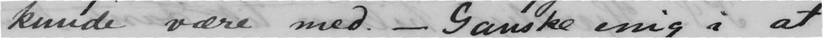kunde være med. -- Ganske enig i at
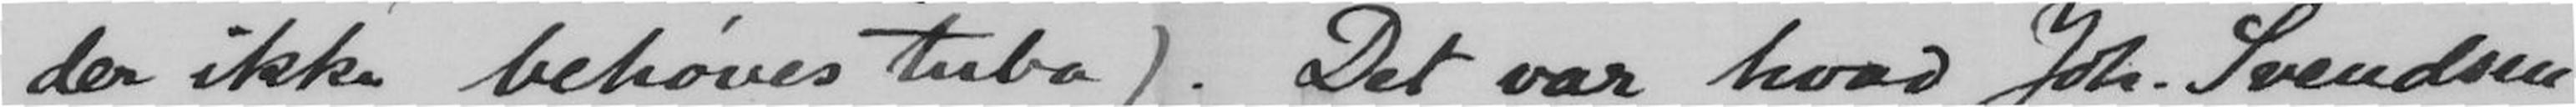der ikke behøves tuba). Det var hvad Joh. Svendsen
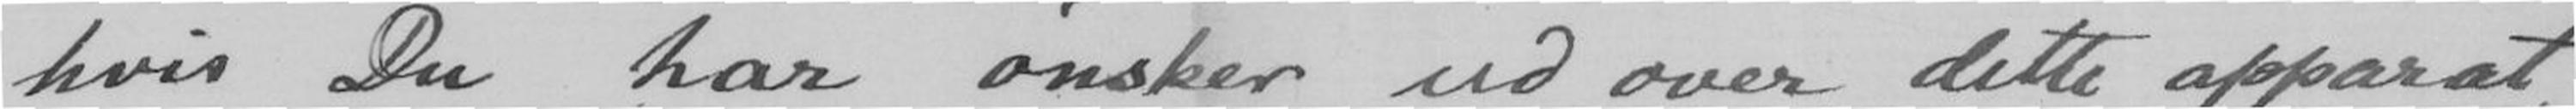hvis Du har ønsker ud over dette apparat,
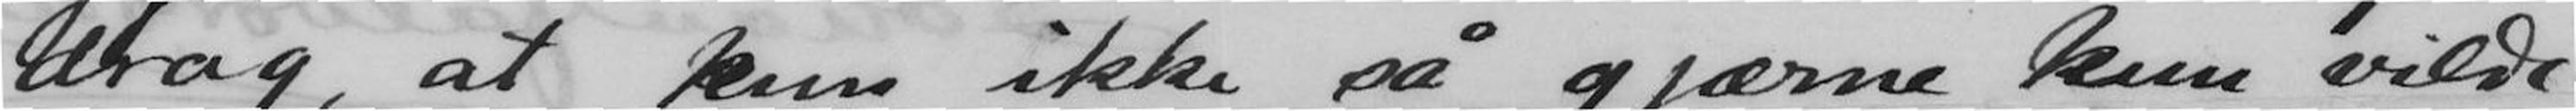drag, at hun ikke så gjærne hun vilde
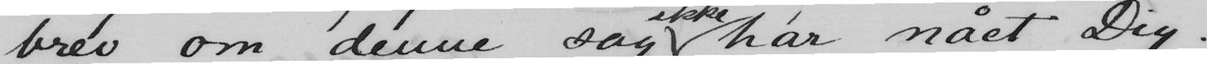brev om denne sag har nået Dig.
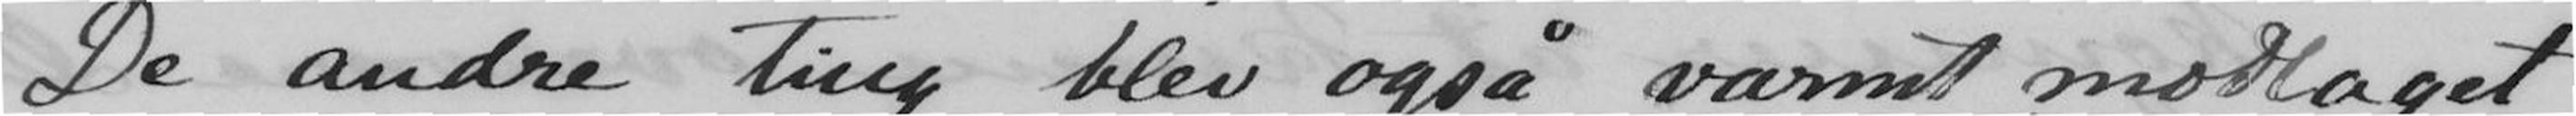De andre ting blev også varmt modtaget
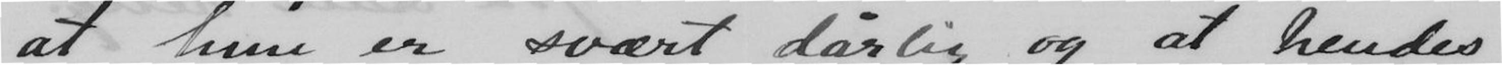at hun er svært dårlig og at hendes
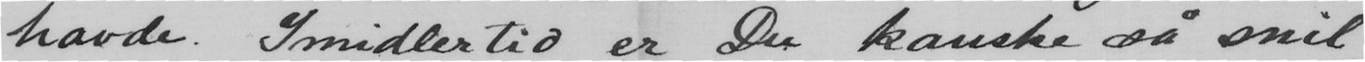havde. Imidlertid er Du kanske så snil
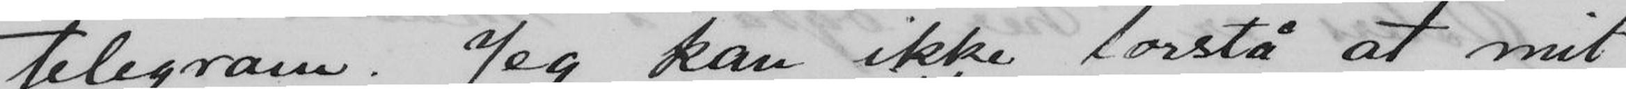telegram. Jeg kan ikke forstå at mit
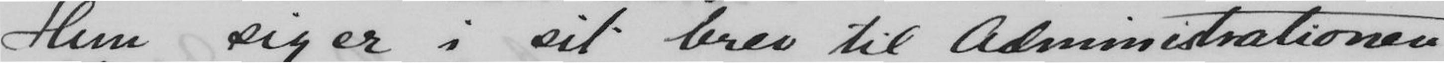Hun siger i sit brev til Administrationen
